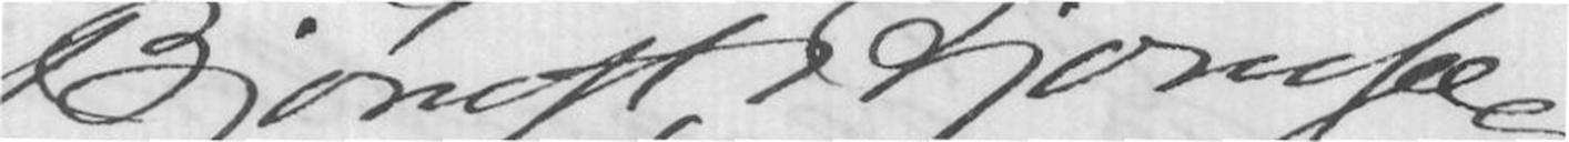Bjørnst, Bjørnson
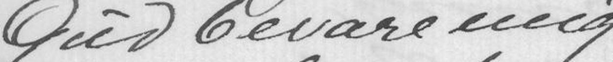Gud bevare mig!
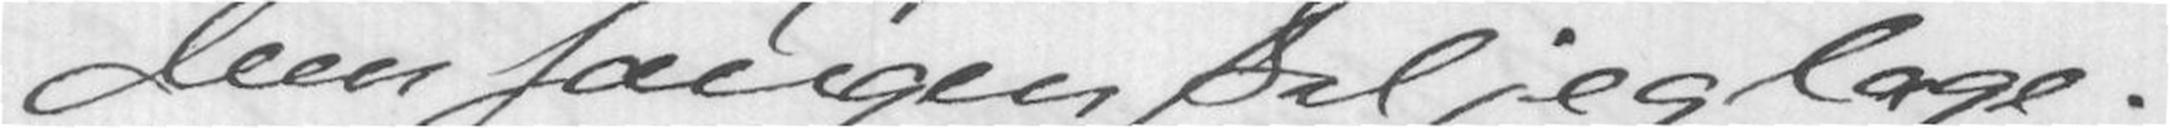Men sangen skal jeg lage.
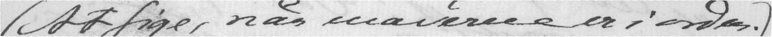(at sige, når maverne er i orden.)
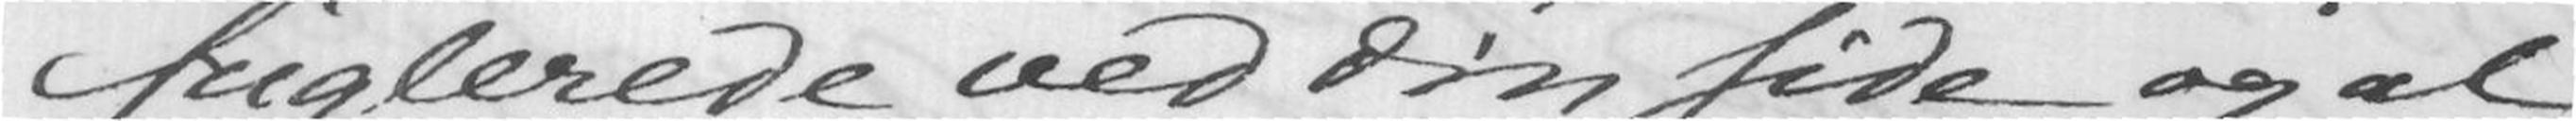fuglerede ved din side og al
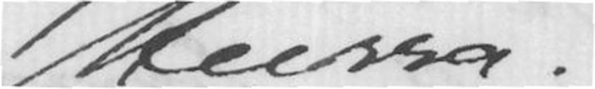Hurra!
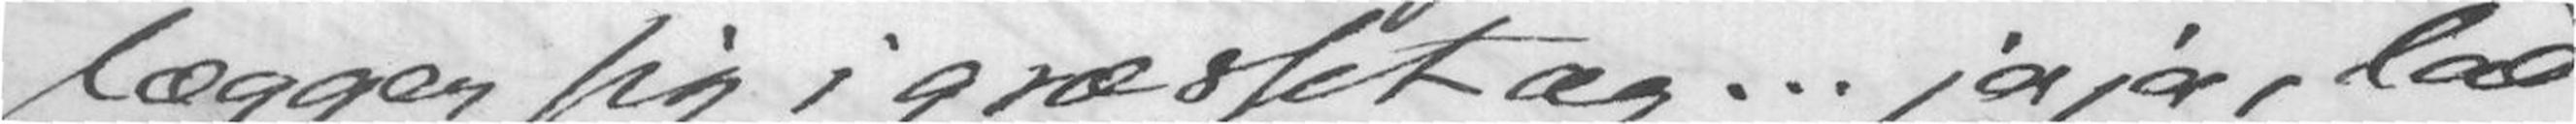lægger sig i græsset og... ja ja,
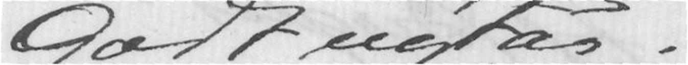Godt nytår!
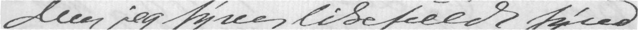Men jeg synes likefuldt synd
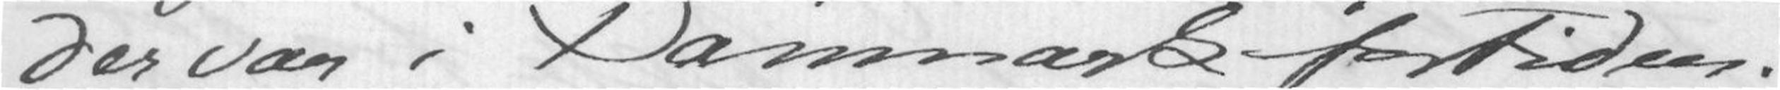der var i Danmark fortiden.
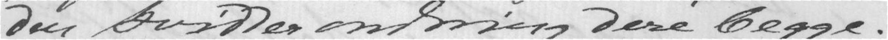den kvidder omkring dere begge.
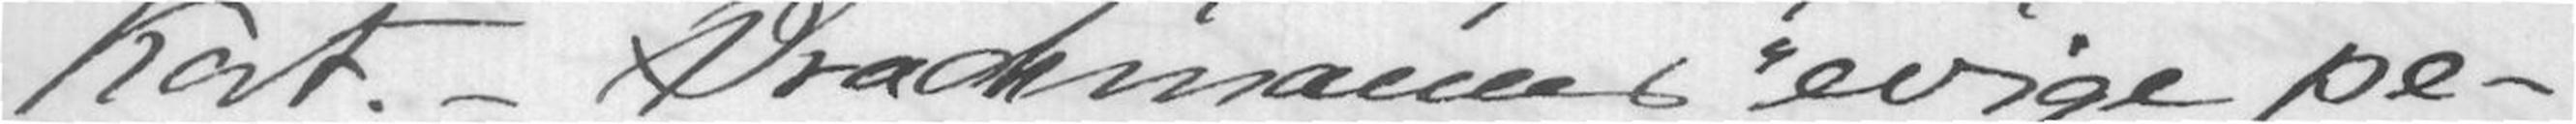Kort. - Drachmanns evige pe-
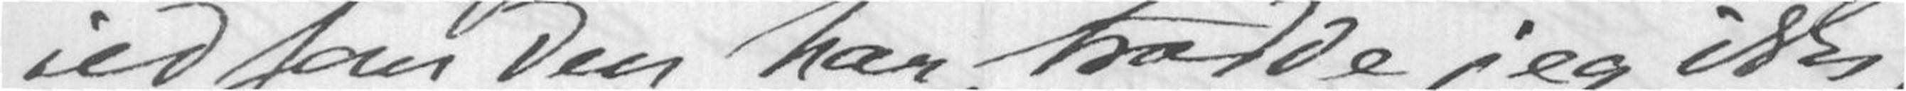ild som den har, trodde jeg ikke,
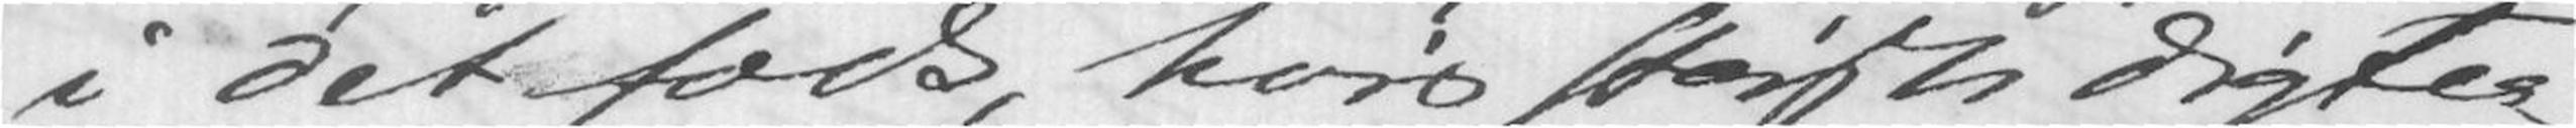i det folk, hvis største digter
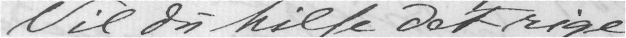Vil du hilse det rige
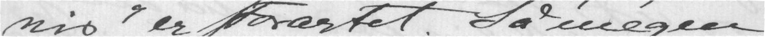nis er storartet. Så megen
